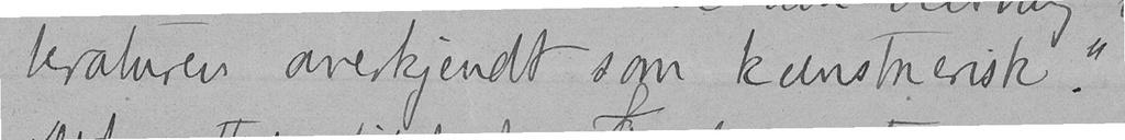teraturen anerkjendt som kunstnerisk."
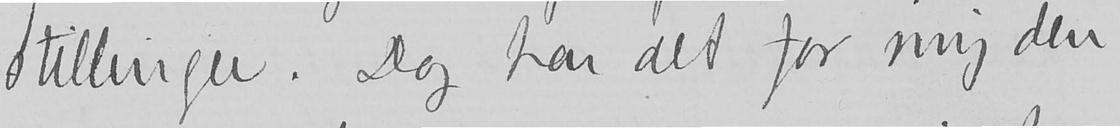stillinger. Dog har det for mig den
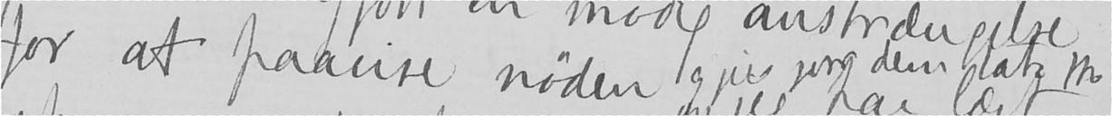for at paavise nøden og pi sorg dem lak M
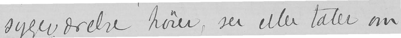sygeværelse hører, ser eller taler om
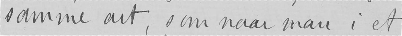samme art, som naar man i et
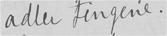adler tingene.
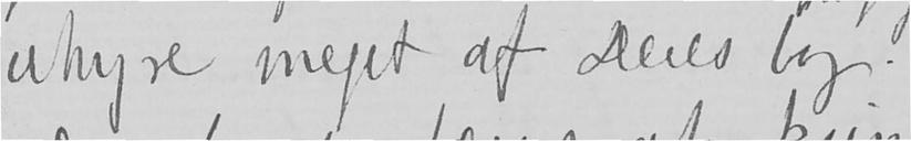uhyre meget af Deres bog.
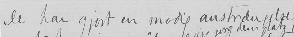De har gjort en modig anstrængelse
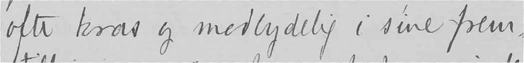ofte kras og modbydelig i sine frem-
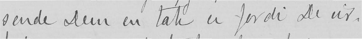sende Dem en tak er fordi De vir-
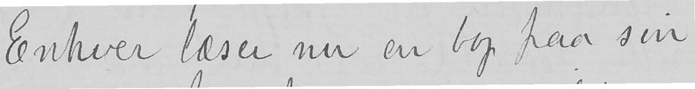Enhver læser nu en bog paa sin
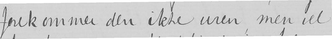forekommer den ikke uren, men vel
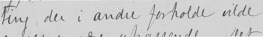ting, der i andre forholde vilde
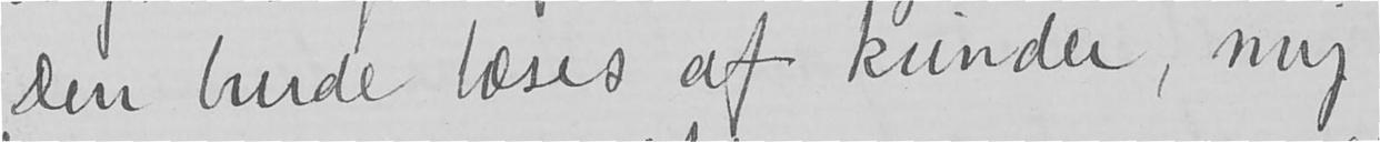Den burde læses af kvinder, mig
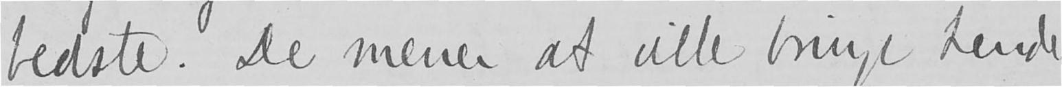bedste. De mener at ville bringe hende
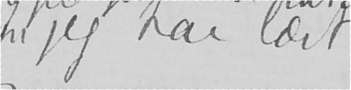som jeg har lært
